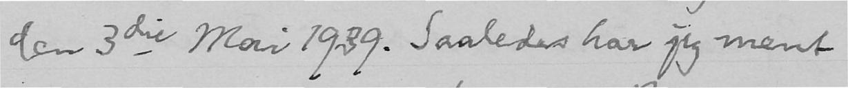den 3die Mai 1939. Saaledes har jeg ment
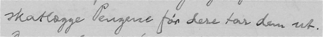skatlægge Pengene før dere tar dem ut.
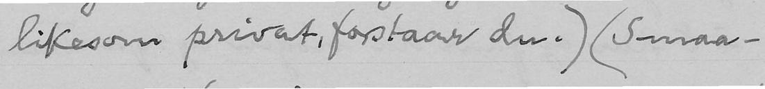likesom privat, forstaar du.) (Smaa-
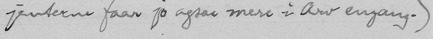jenterne faar jo ogsaa mere i Arv engang.)
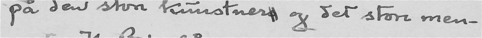på den store kunstners og det store men-
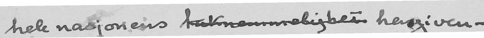hele nasjonens taknemmelighet hengiven-
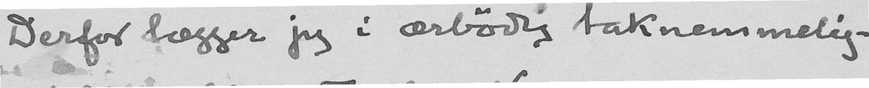Derfor lægger jeg i ærbødig taknemmelig-
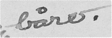båre.
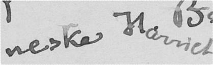neske Harriet
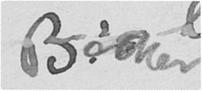Backers
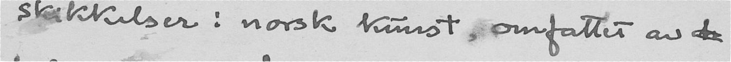skikkelser i norsk kunst, omfattet av h
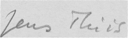Jens Thiis
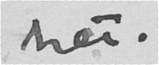het.
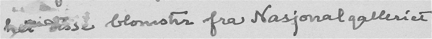het disse blomster fra Nasjonalgalleriet
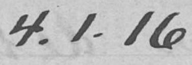4.1.16
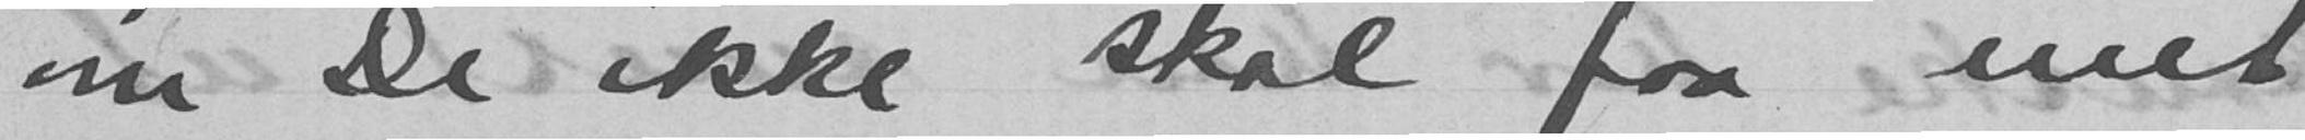om De ikke skal faa mit
